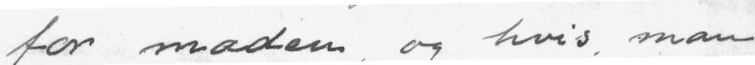for maden, og hvis man
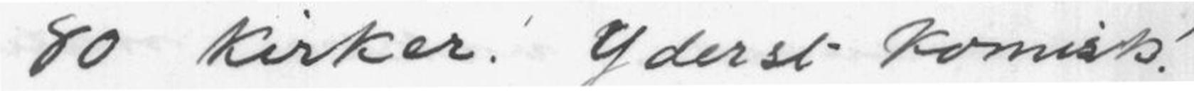80 kirker. Yderst komisk!
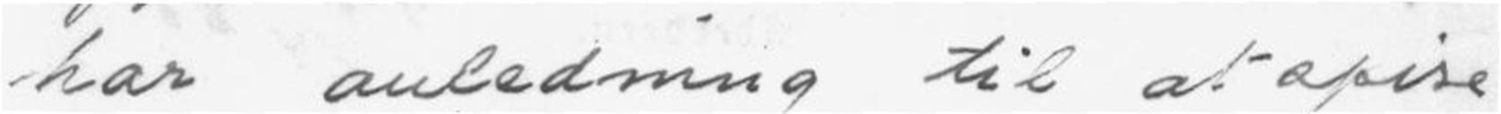har anledning til at spise
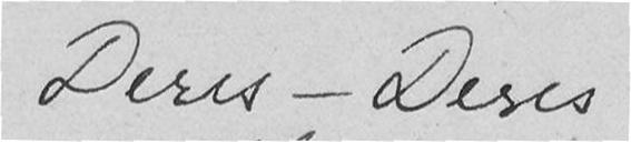Deres - Deres
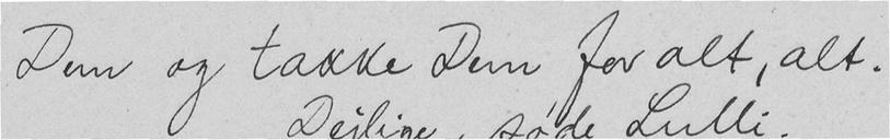Dem og takke Dem for alt, alt.
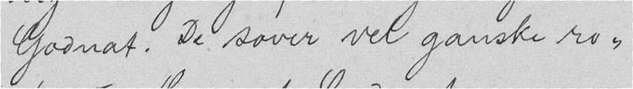Godnat. De sover vel ganske ro-
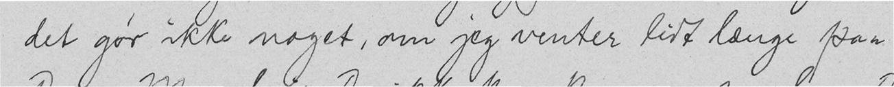det gør ikke noget, om jeg venter lidt længe paa
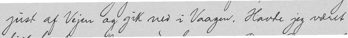just af Vejen og gik ned i Vaagen. Havde jeg været
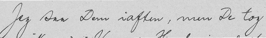Jeg saa Dem iaften, men De tog
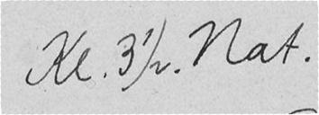Kl. 3½. Nat.
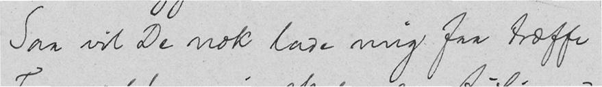Saa vil De nok lade mig faa træffe
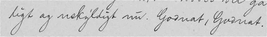ligt og uskyldigt nu. Godnat, Godnat.
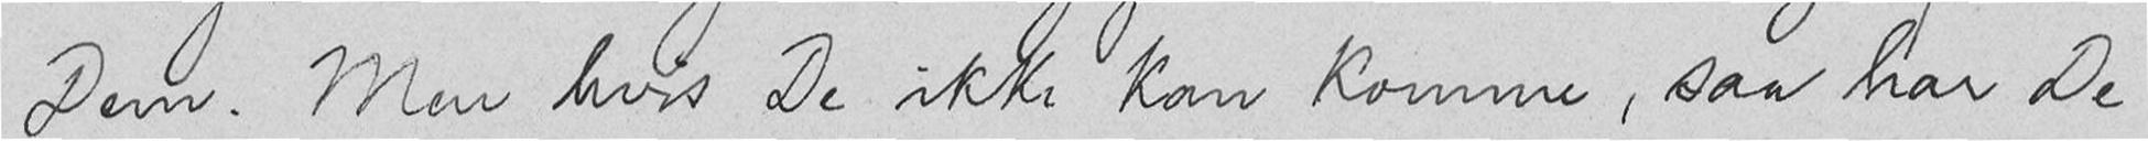Dem. Men hvis De ikke kan komme, saa har De
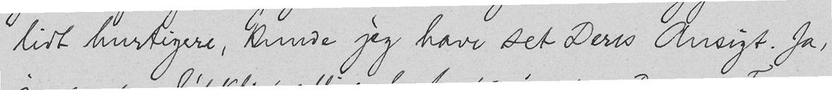lidt hurtigere, kunde jeg have set Deres Ansigt. Ja,
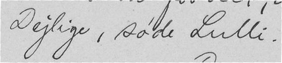Dejlige, søde Lulli.
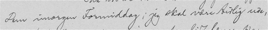Dem imorgen Formiddag; jeg skal være tidlig ude,
